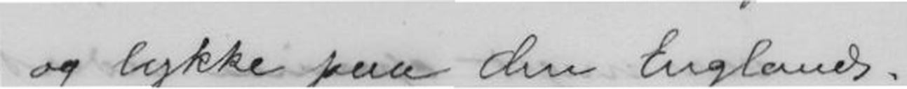og lykke paa din Englands-
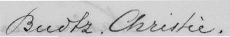Budtz Christie
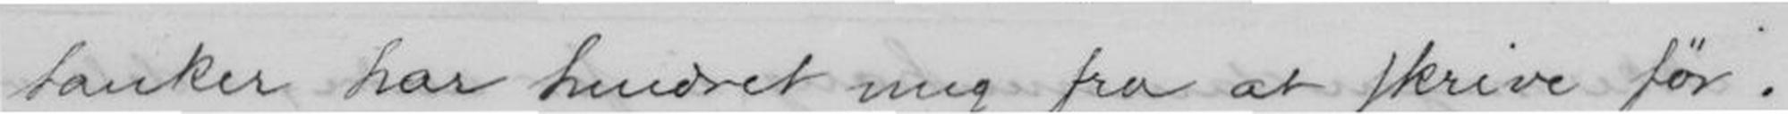tanker har hindret mig fra at skrive før.
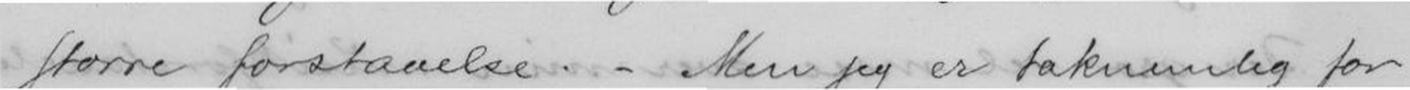større forstaaelse. - Men jeg er taknemlig for
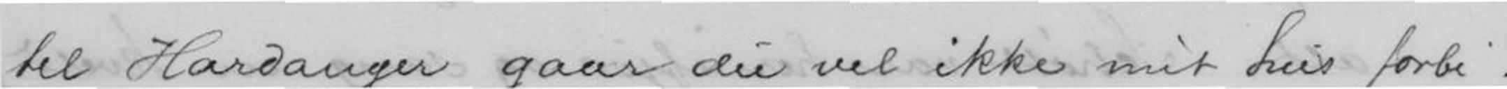til Hardanger gaar du vel ikke mit hus forbi.
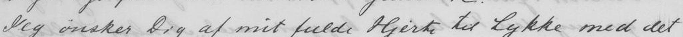Jeg ønsker Dig af mit fulde Hjerte til lykke med det
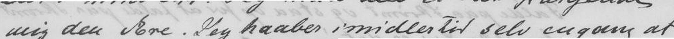mig den Ære. Jeg haaber imidlertid selv engang at
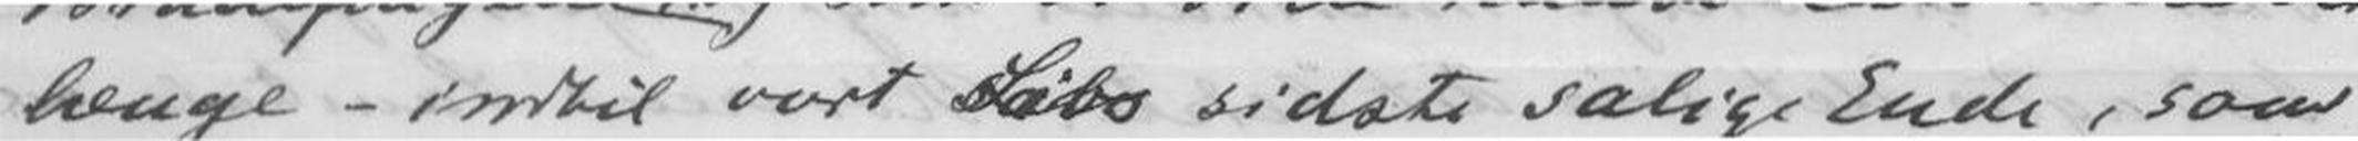længe - indtil vort Livs sidste salige Ende, som
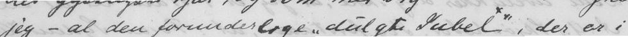jeg - al den forundelige "dulgte Jubelx", der er i
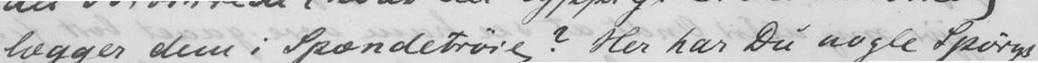lægger dem i Spændetrøie Her har Du nogle Spørgs-
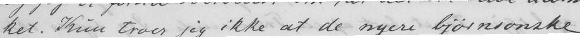ket. Kun troes jeg ikke at de nyere bjørnsonske
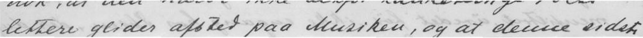lettere glider afsted paa Musiken, og at denne sidste
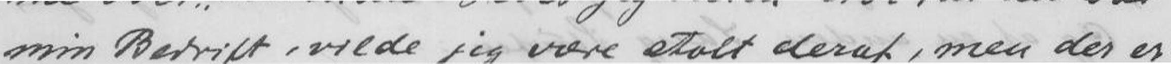min Bedrift, vilde jeg være stolt deraf, men der er
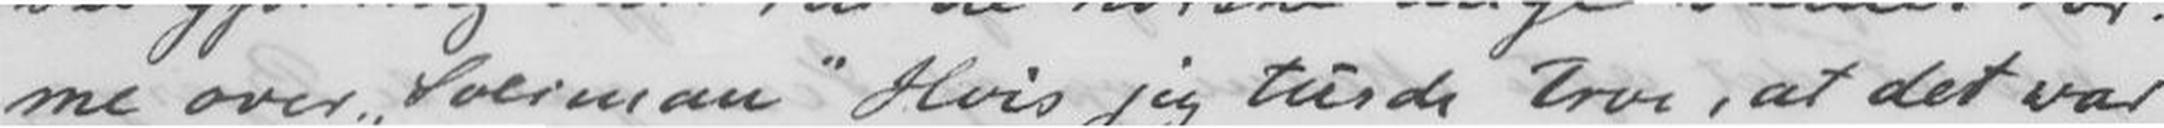me over Soliman Hvis jeg turde troe, at det var
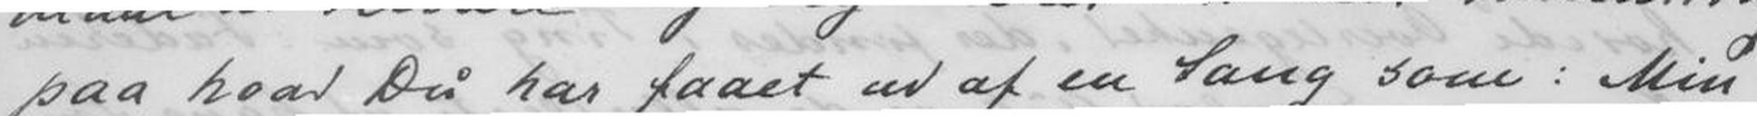paa hvad Du har faaet ud af en Sang som: Min
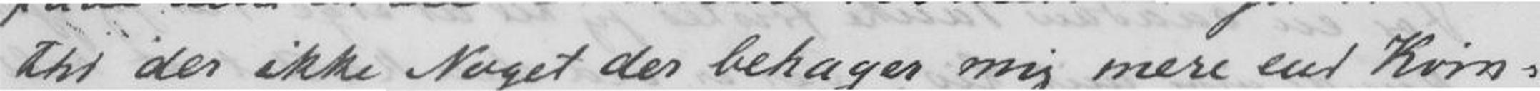thi der ikke Noget der behager mig mere end Kvin-
